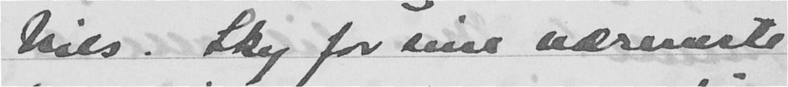Nils. Sky for sine nærmeste
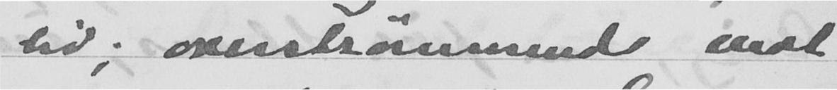liv; overstrømmende mot
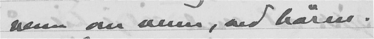venn om veien, ved båren.
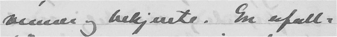venner og bekjente. En ufull-
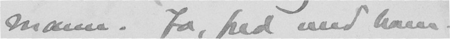mann. Ja, fred med ham.
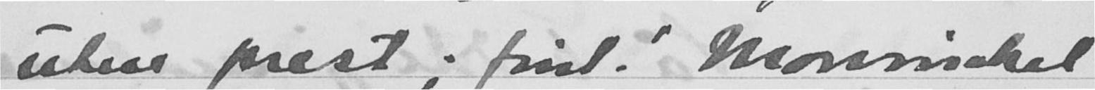uten prest; fint! Mowinckel
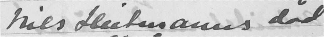Nils Hirtmanns død
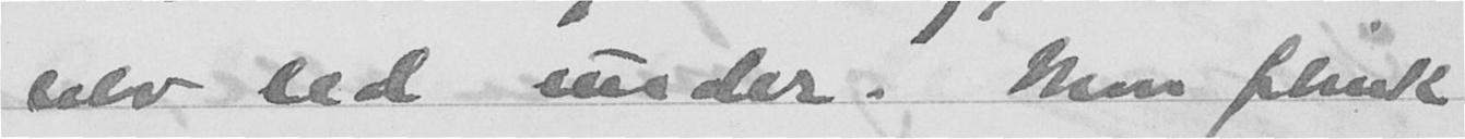selv led under. Men flink
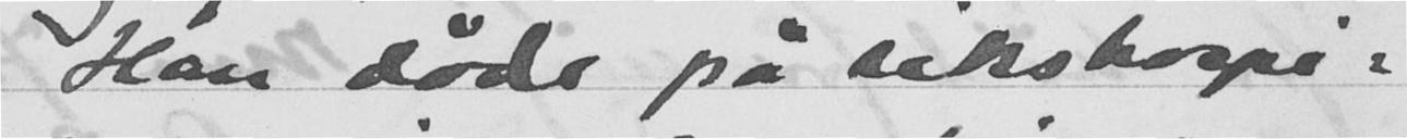Han døde på rikshospi-
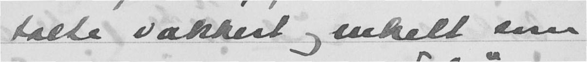talte vakkert og enkelt som
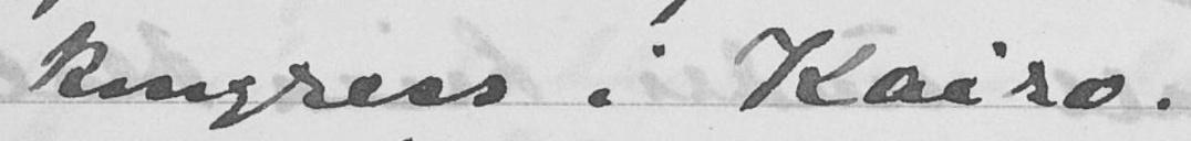kongress i Kairo.
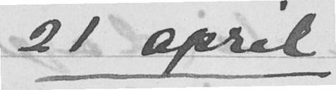21 april
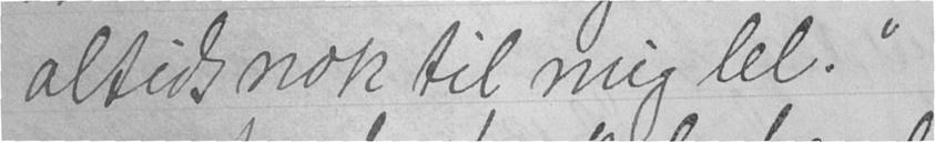altidsnok til mig lel."
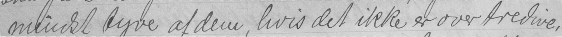mindst tyve af dem, hvis det ikke er over tredive,
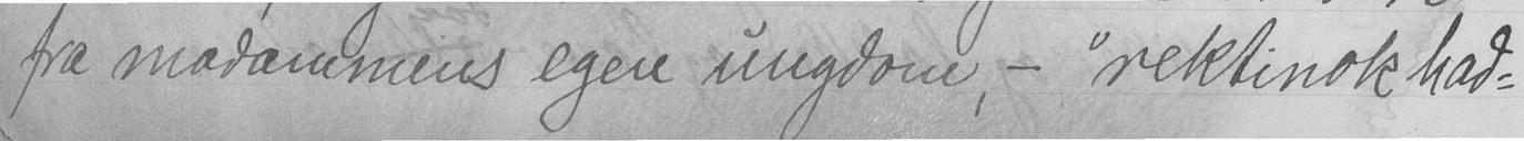fra madammens egen ungdom, - "rektinok had-
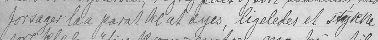forsager laa parat til at syes, ligeledes et stykke
